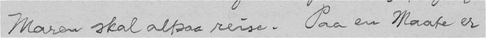Maren skal altsaa reise. Paa en Maate er
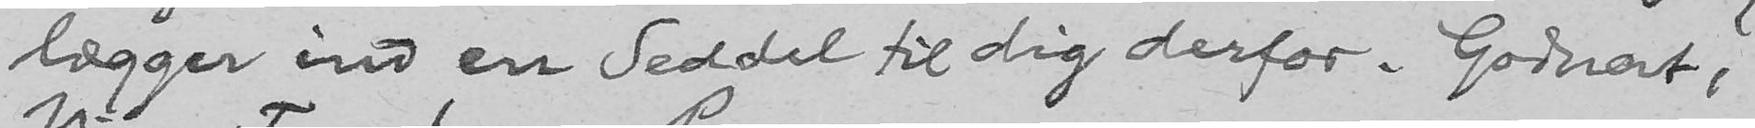lægger ind en Seddel til dig derfor. Godnat,
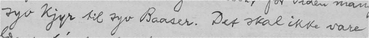syv Kjyr til syv Baaser. Det skal ikke vare
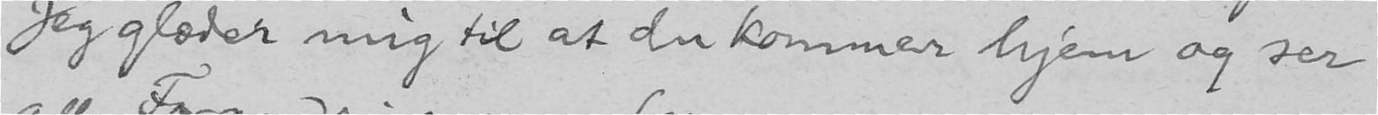Jeg glæder mig til at du kommer hjem og ser
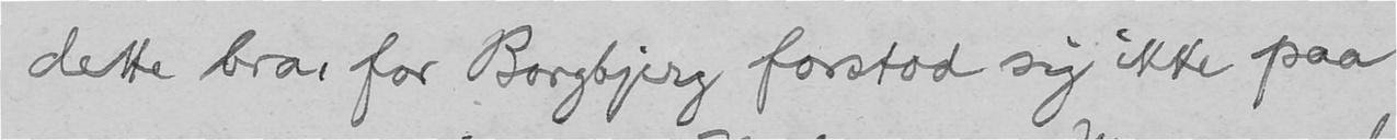dette bra, for Borgbjerg forstod sig ikke paa
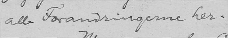alle Forandringerne her.
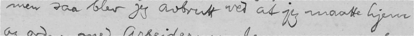men saa blev jeg avbrutt ved at jeg maatte hjem
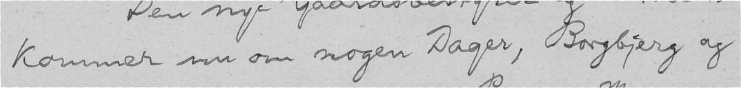kommer nu om nogen Dager, Borgbjerg og
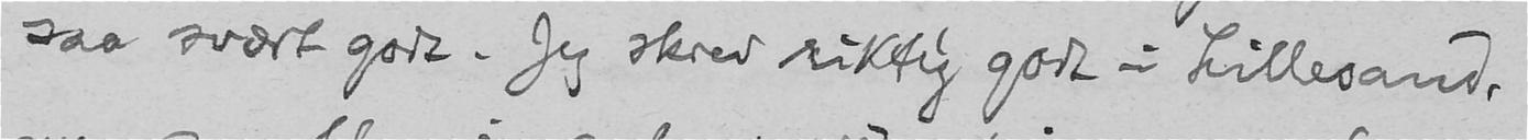saa svært godt. Jeg skrev riktig godt i Lillesand,
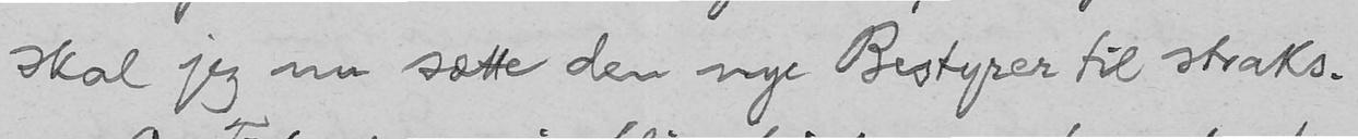skal jeg nu sætte den nye Bestyrer til straks.
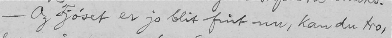- Og Fjøset er jo blit fint nu, kan du tro,
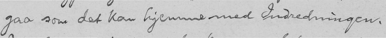gaa som det kan hjemme med Indredningen.
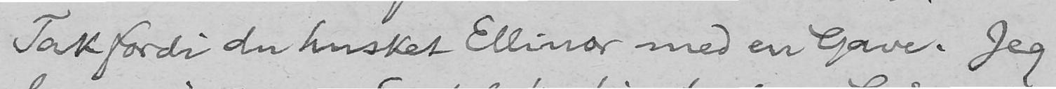Tak fordi du husket Ellinor med en Gave. Jeg
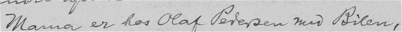Mama er hos Olaf Pedersen med Bilen,
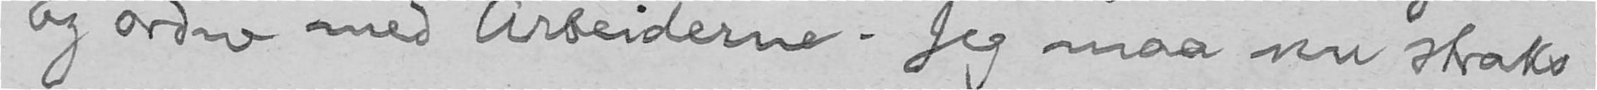og ordne med Arbeiderne. Jeg maa nu straks
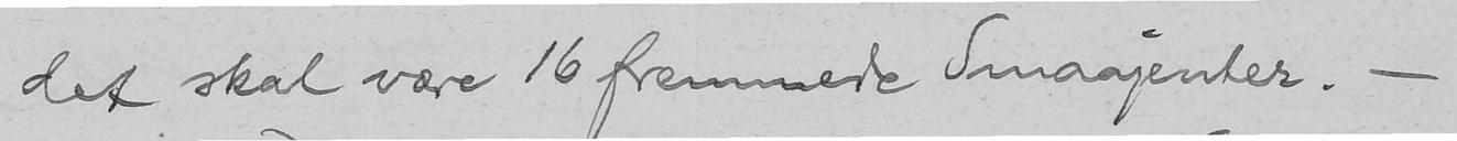det skal være 16 fremmede Smaajenter. -
